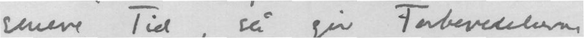senere Tid, så giv Forberedelserne
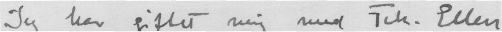Jeg har giftet mig med Frk. Ellen
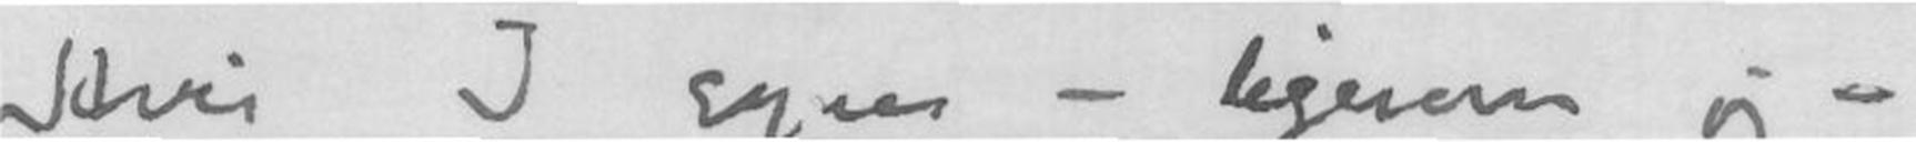Hvis I synes - ligesom jeg -
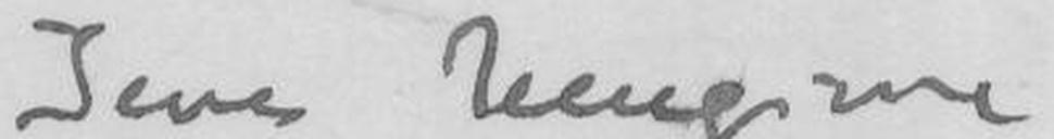Deres hengivne
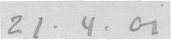21.4.01
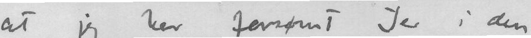at jeg har forsømt Jer i den
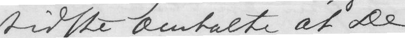sidste omtalte at De
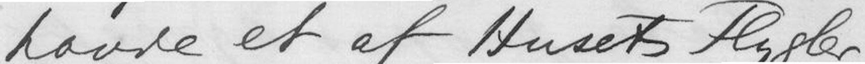havde et af Husets Flygler,
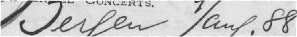Bergen 9/aug. 88
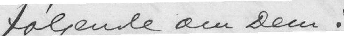følgende om Dem:
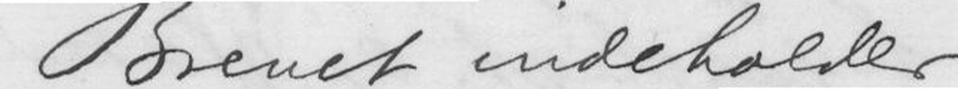- Brevet indeholder
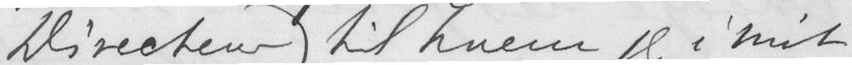Directeur) til hvem jeg i mit
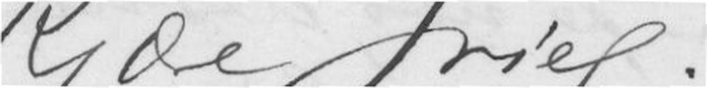Kjære Grieg.
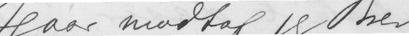Igaar modtog jeg Brev
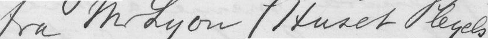fra Mr Lyon (Huset Plyels
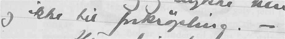og ikke til forkrøpling. -
